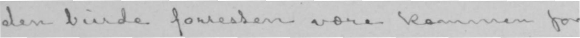den burde forresten være kommen for
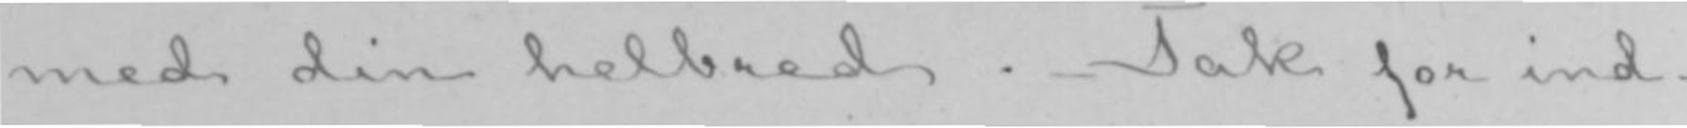med din helbred. Tak for ind-
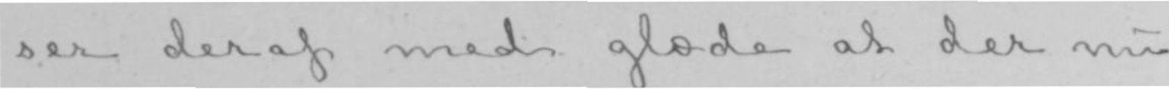ser deraf med glæde at der nu
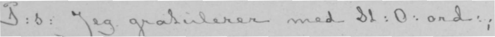P: s: Jeg gratulerer med St: O: ord:;
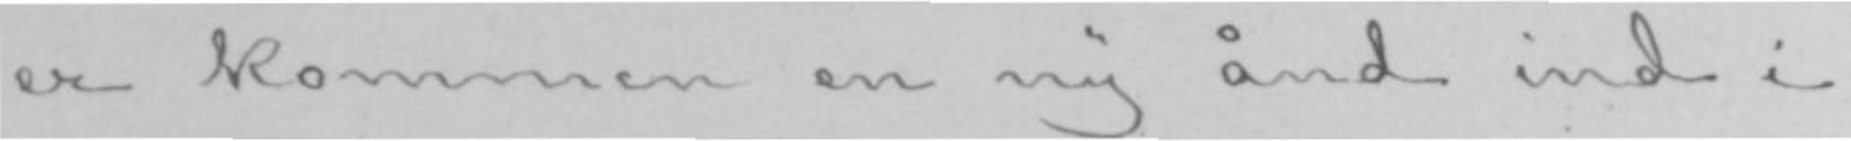er kommen en ny ånd ind i
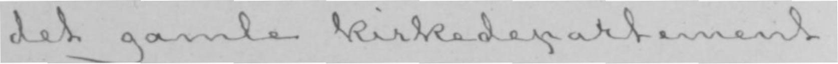det gamle kirkedepartement.
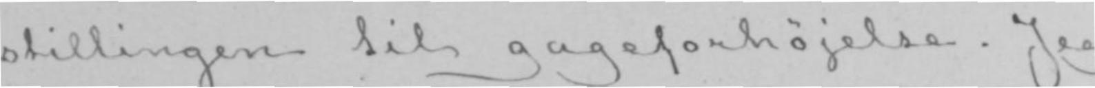stillingen til gageforhøjelse. Jeg
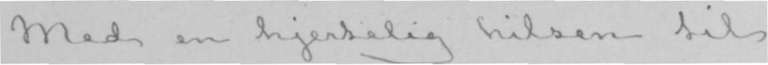Med en hjertelig hilsen til
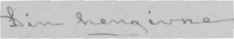Din hengivne
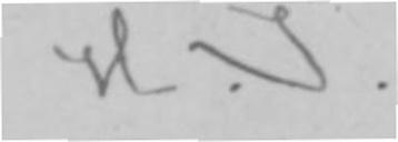H. I.
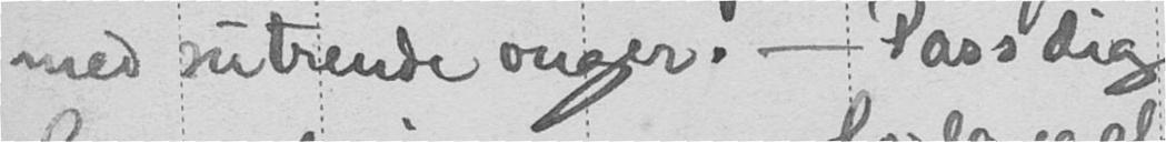med sutrende ouger. - Pass dig
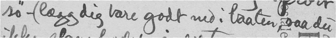sø - (lægg dig bare godt ned i baaten, saa du
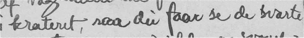i krateret saa du faar se de svarte
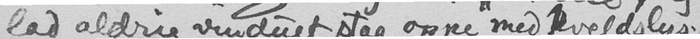lad aldrig vinduet staa oppe med kveldslys.
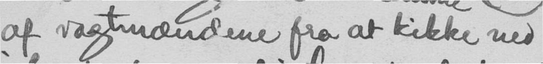af vagtmændene fra at kikke ned
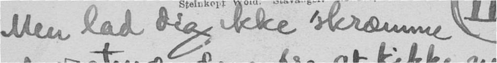Men lad dig ikke skræmme
